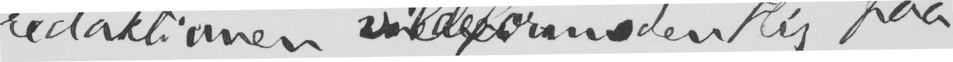redaktionen vilde formodentlig paa
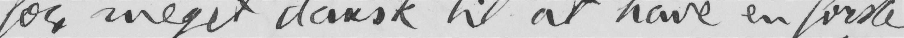for meget dansk til at have en første
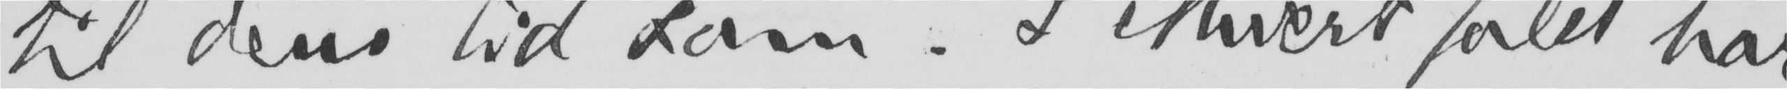til dens tid kom. I ethvert fald har
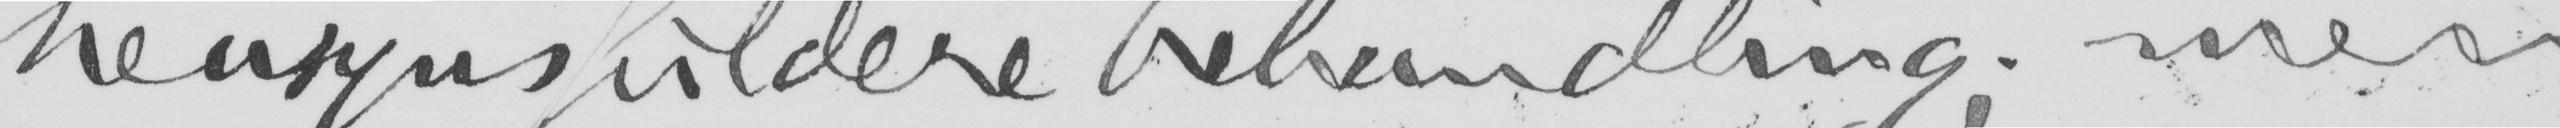hensynsfuldere behandling; men
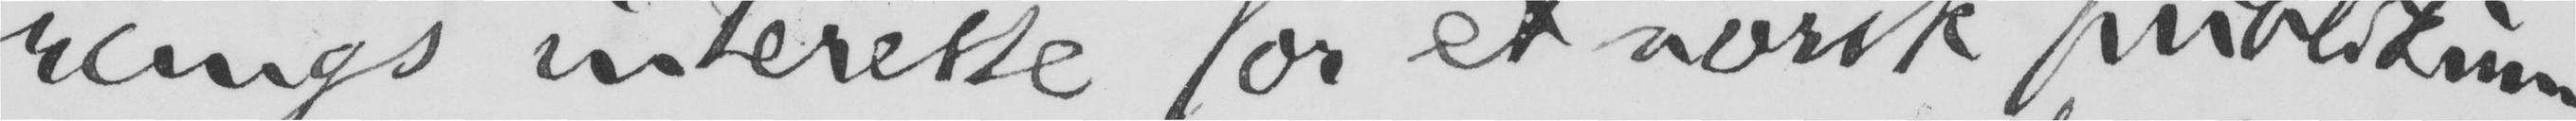rangs interesse for et norsk publikum,
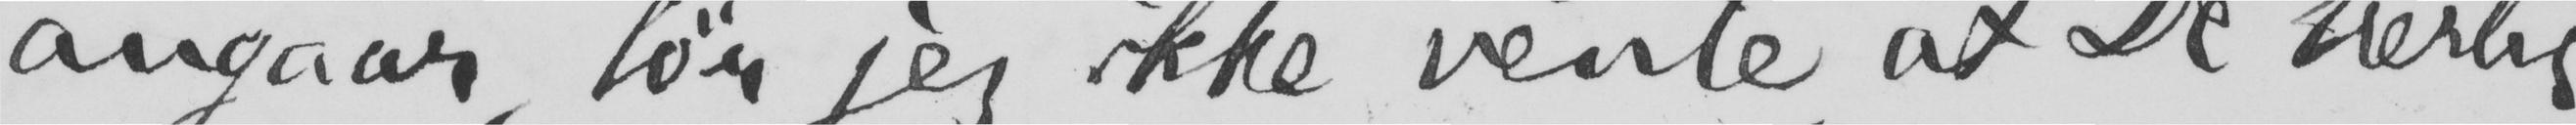angaar, tør jeg ikke vente at De særlig
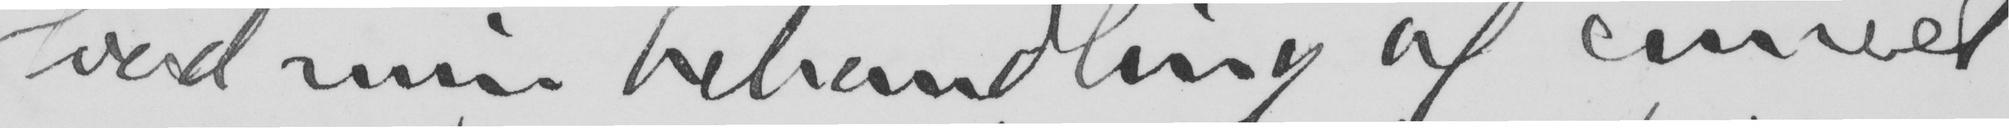Hvad min behandling af emnet
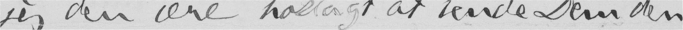jeg den ære hoslagt at sende Dem den
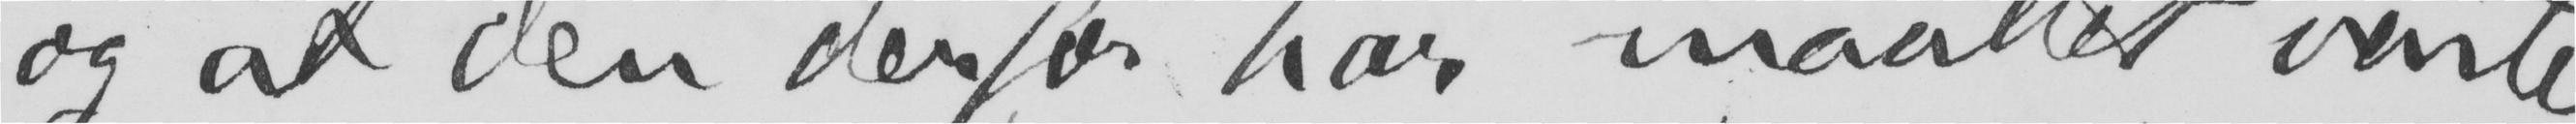og at den derfor har maattet vente,
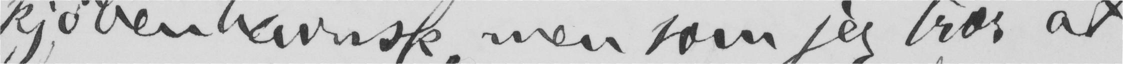kjøbenhavnsk, men som jeg tror at
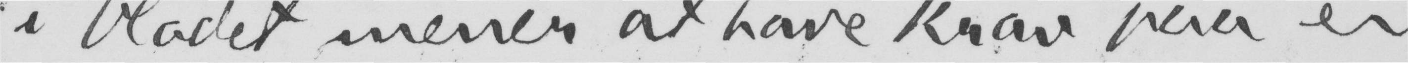i bladet mener at have krav paa en
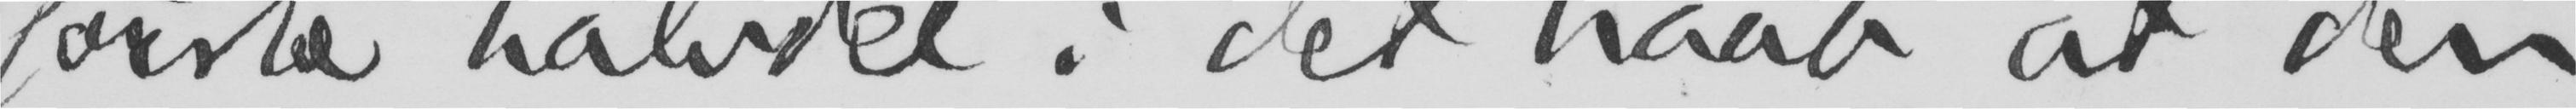første halvdel i det haab, at den
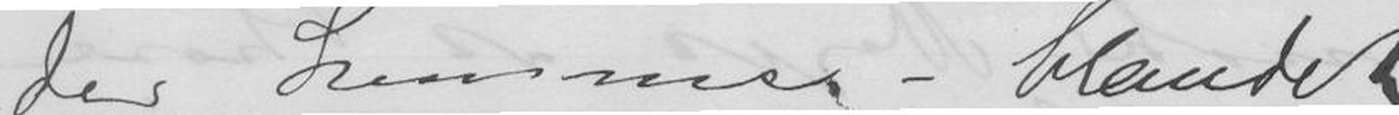der komme - blandet
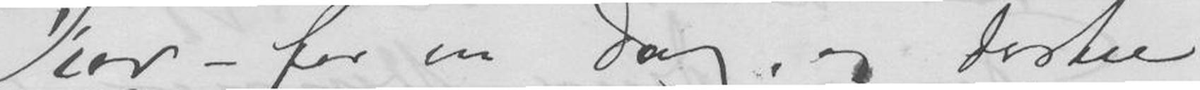Kor - for en Dag og dertil
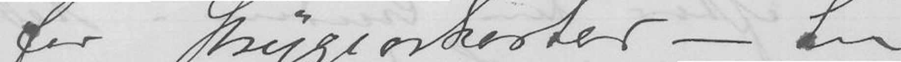for Strygeorkester - til
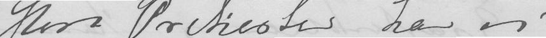stort Orchester har vi
